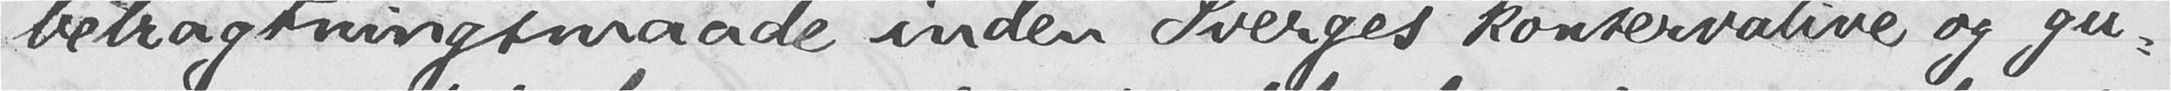betragtningsmaade inden Sverges konservative og gu-
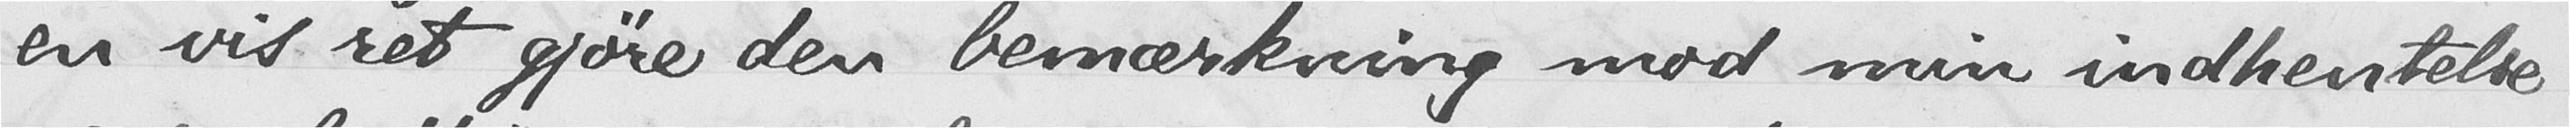en vis ret gjøre den bemærkning mod min indhentelse
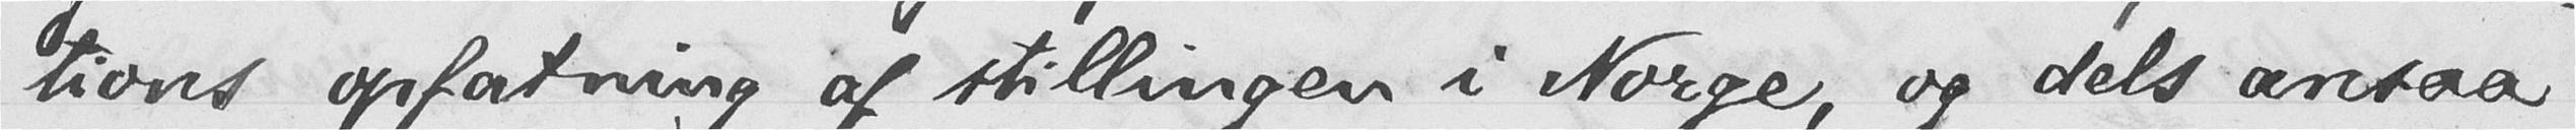tions opfatning af stillingen i Norge, og dels ansaa
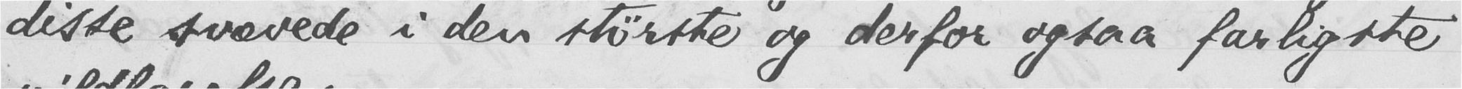disse svævede i den største og derfor ogsaa farligste
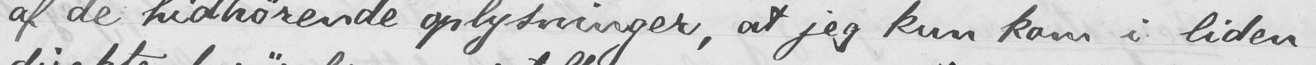af de hidhørende oplysninger, at jeg kun kom i liden
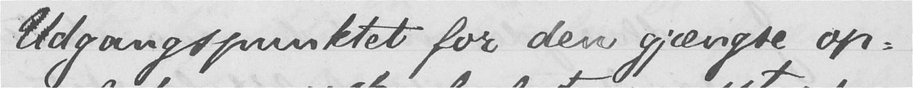Udgangspunktet for den gjængse op-
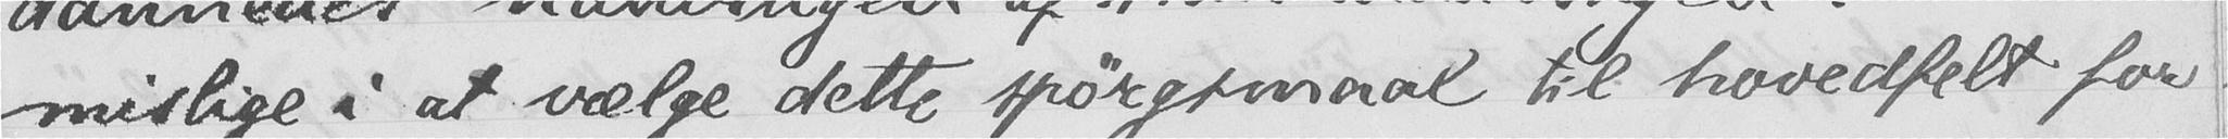mislige i at vælge dette spørgsmaal til hovedfelt for
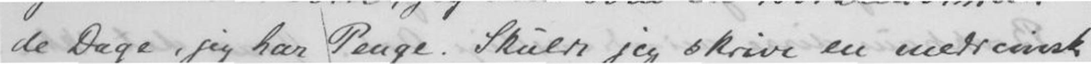de Dage jeg har Penge. Skulde jeg skrive en medicinsk
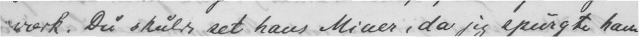værk. Du skulde set hans Miner, da jeg spurgte ham
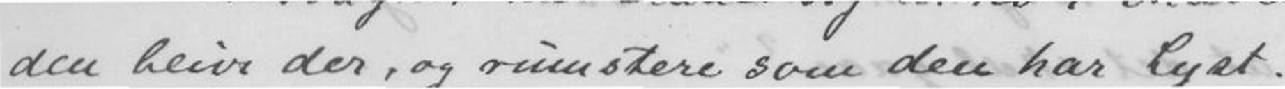den blive der, og rumstere som den har Lyst.
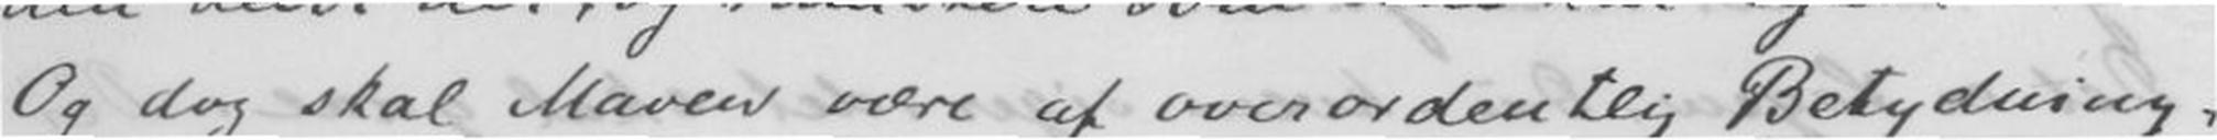Og dog skal Maven være af overordenlig Betydning
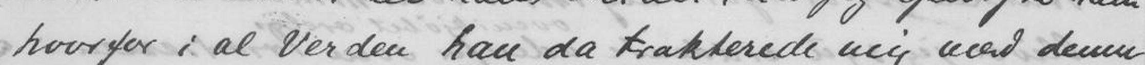hvorfor i al Verden han da traterede mig med denne
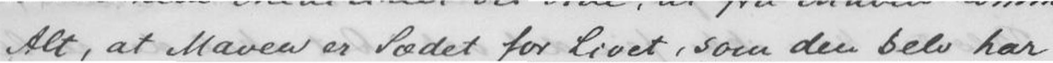Alt, at Maven er Sædet for Livet, som den selv har
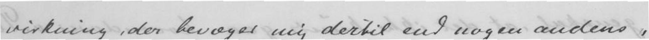virkning der bevæger mig dertil end nogen andens,
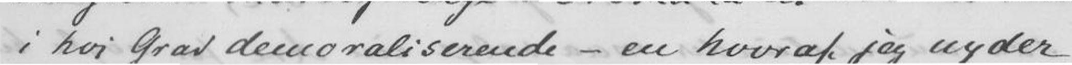i høi Grad demoraliserende - en hvoraf jeg nyder
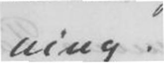ning.-
